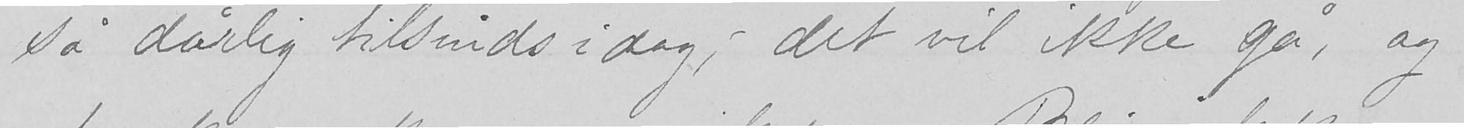så dårlig tilsinds i dag, - det vil ikke gå, og
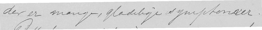der er mange, glædelige symptomer.
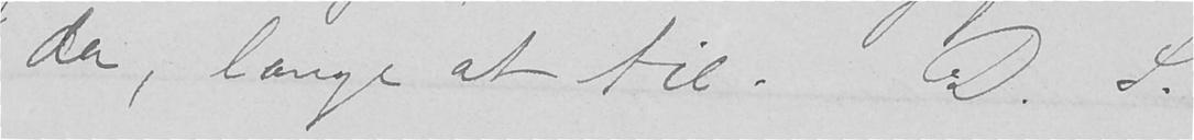da, længe at tie. D. S.
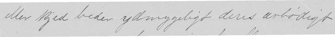eller kjed beder ydmygeligt deres ærbødigt
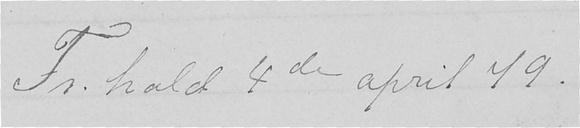Fr. hald 4de april 79.
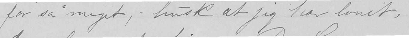for så meget, - husk at jeg har lovet,
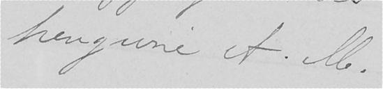hengivne A. M.
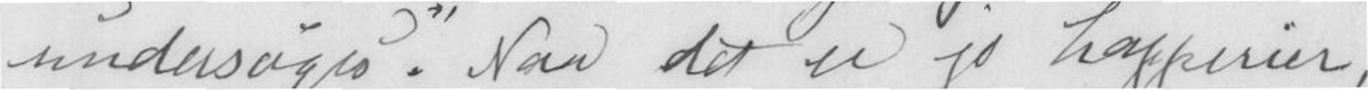undersøges. Naa det er jo Lapperier,
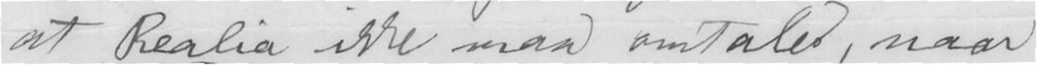at Realia ikke maa omtales, naar
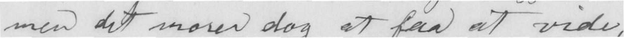men det morer dog at faa at vide,
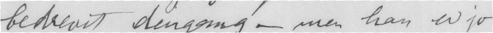bedrevet dengang - men han er jo
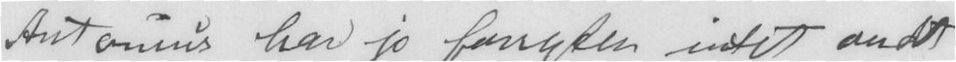Antonius har jo forresten intet ondt
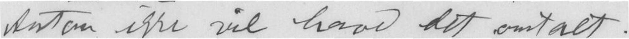Anton ikke vil have det omtalt.
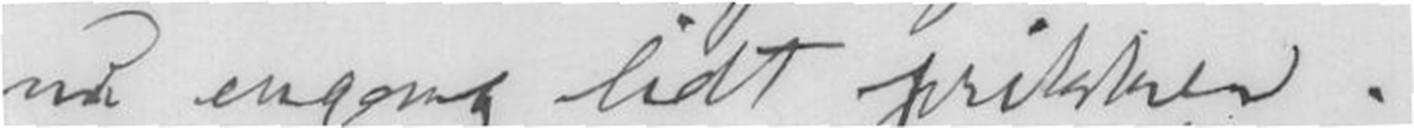nu engang lidt prikken:
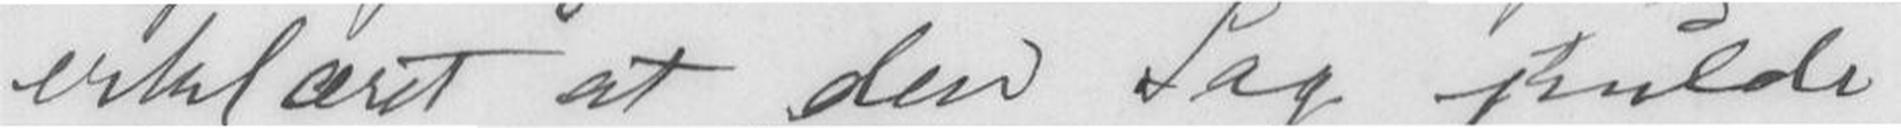erklæret at den Sag skulde
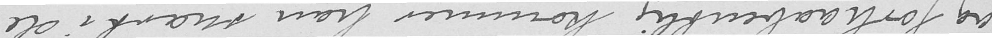og forhaabentlig kommer han snart i de
